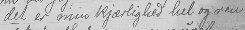det er min kjærlighed hel og ren
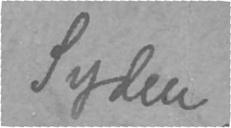lyden
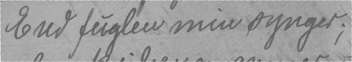End fuglen min synger;
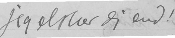Jeg elsker dig end!
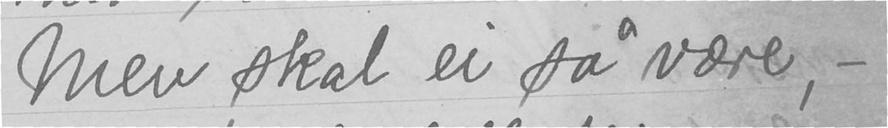Men skal ei så være, -
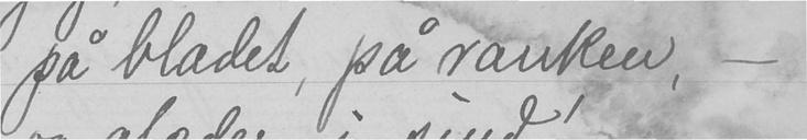på bladet, på ranken, -
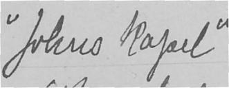"Johns Kapel"
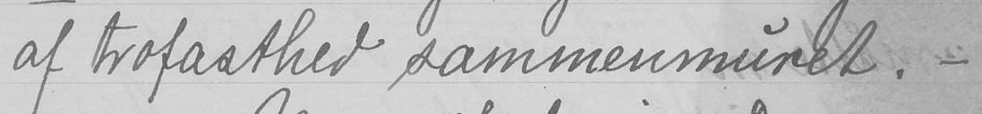af trofasthed sammenmuret. -
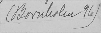(Bornholm 96)
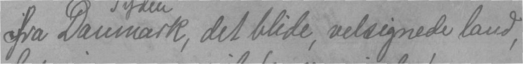fra Danmark, det blide, velsignede land,
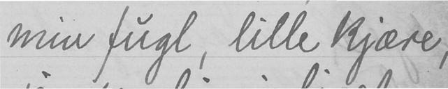min fugl, lille kjære,
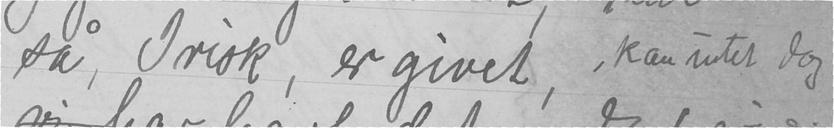så, Irisk, er givet, kan intet dog
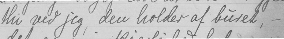thi ved jeg, den holder af buret, -
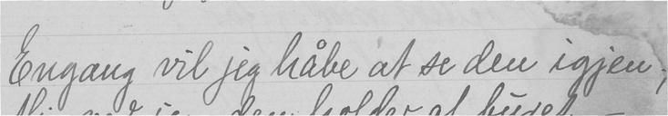Engang vil jeg håbe at se den igjen;
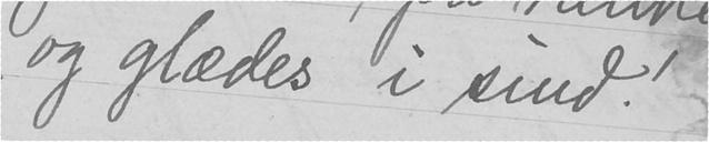og glædes i sind!
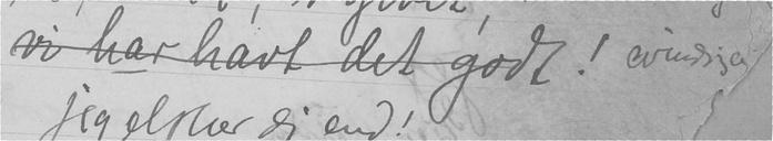vi har havt det godt!
